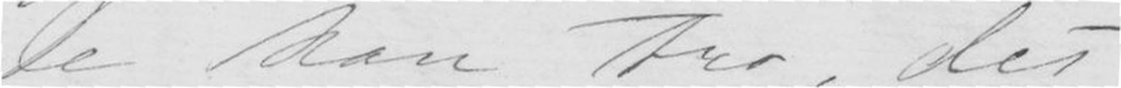De kan tro, det
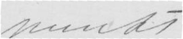punkt.
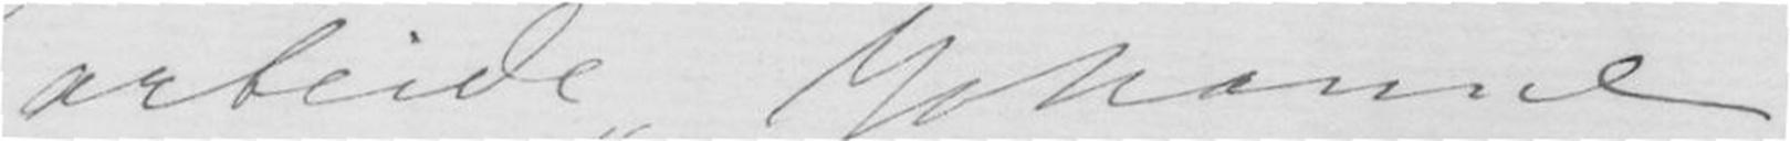arbeide Johanne.
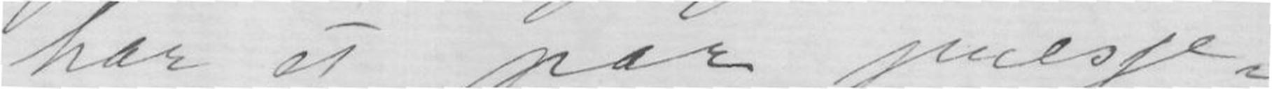har et par presse-
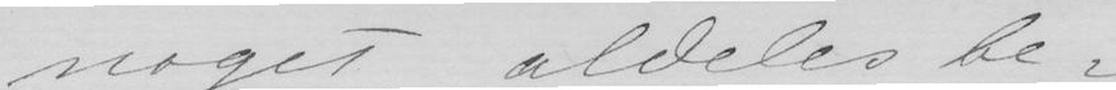noget aldeles be-
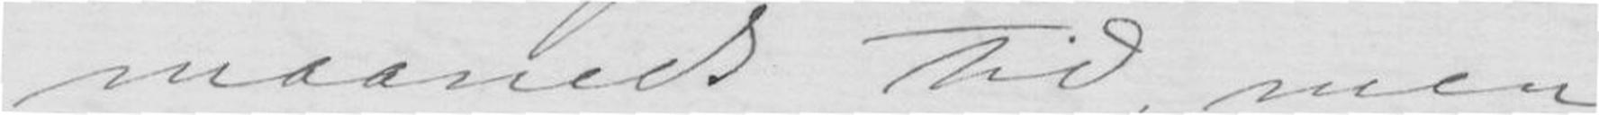maaneds Tid, men
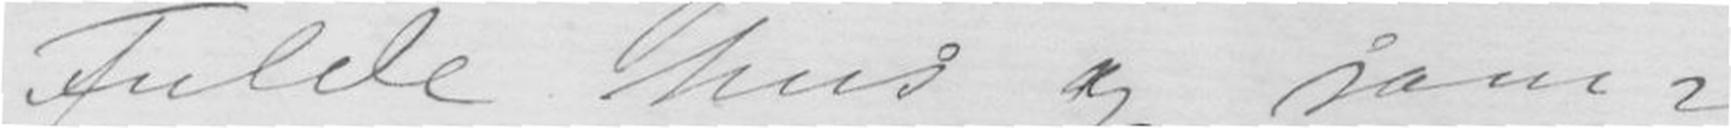Fulde hus og sam-
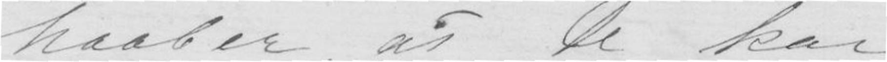haaber, at De kan
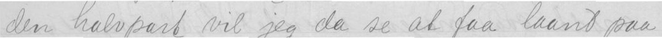den halvpart vil jeg da se at faa laant paa
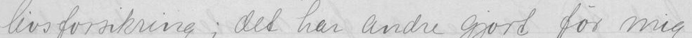livsforsikring; det har andre gjort før mig,
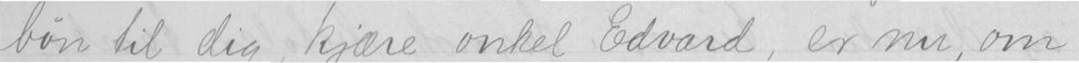bøn til dig, kjære onkel Edvard, er nu, om
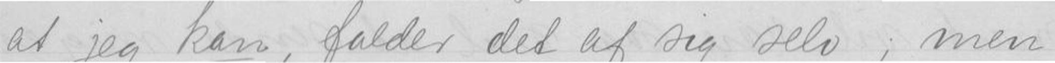at jeg kan, falder det af sig selv; men
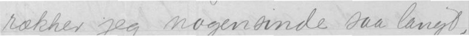rækker jeg nogensinde saa langt,
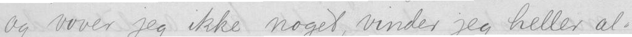og vover jeg ikke noget, vinder jeg heller al-
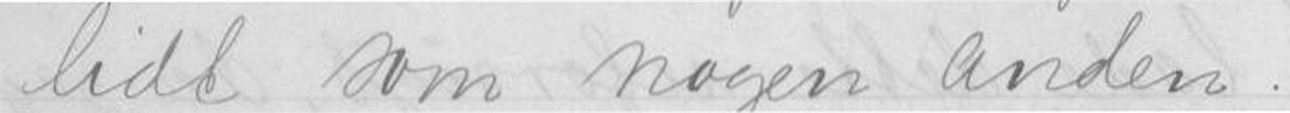lidt som nogen anden.
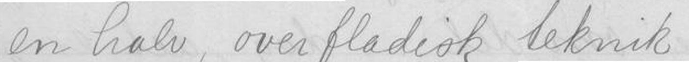en halv overfladisk teknik.
